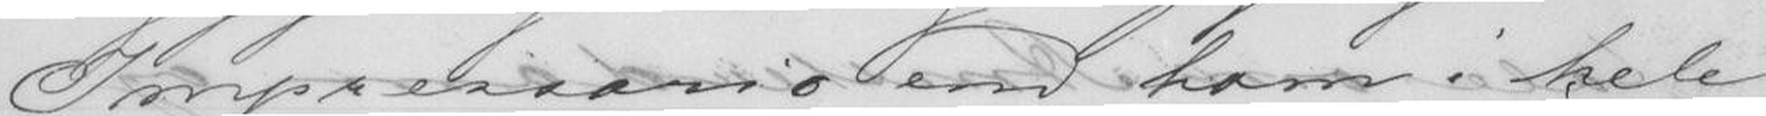Impressario end ham i hele
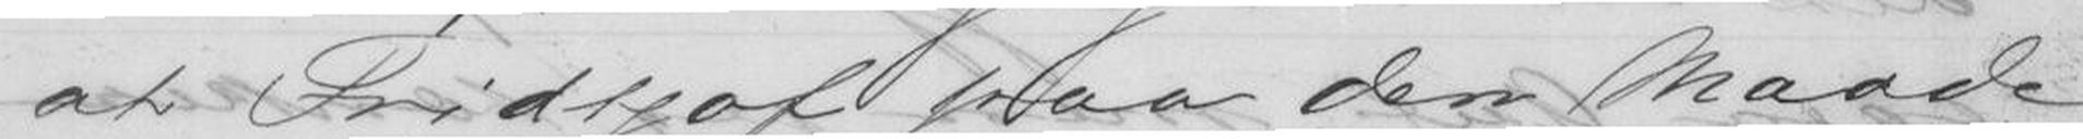at Fridtjof paa den Maade
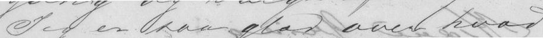Jeg er saa glad over hvad
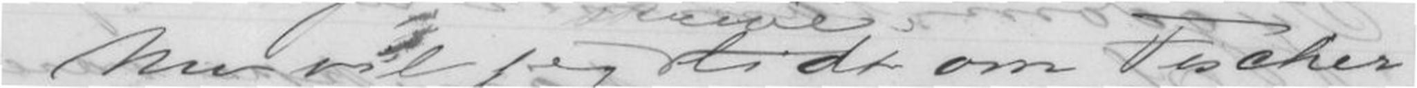Nu vil jeg lidt om Fischer
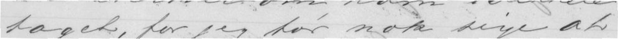taget, for jeg tør nok sige at
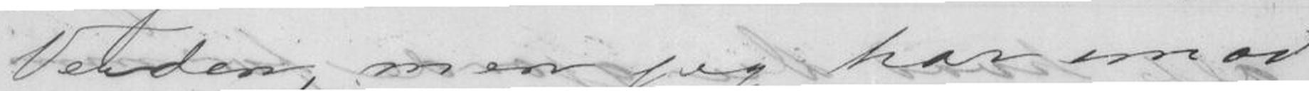Verden, men jeg har imod
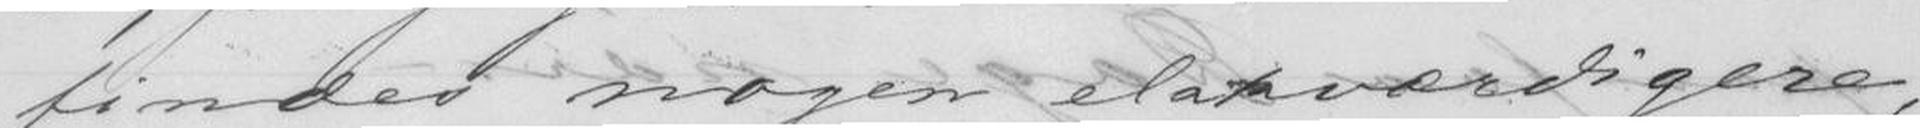findes nogen elskværdigere,
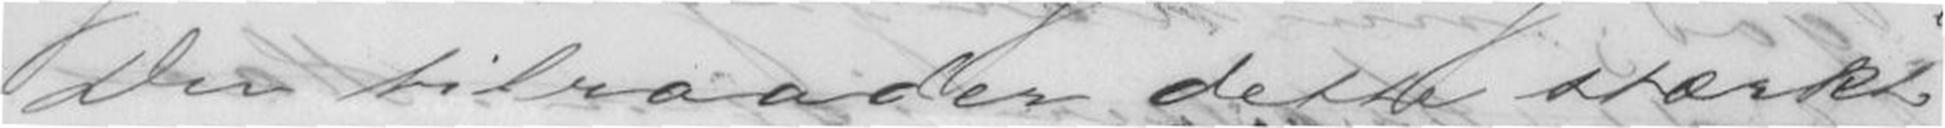Du tilraader dette stærkt
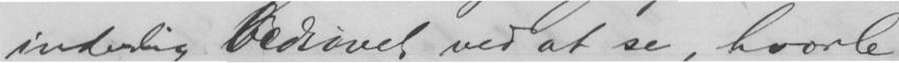inderlig bedrøvet ved at se, hvorle-
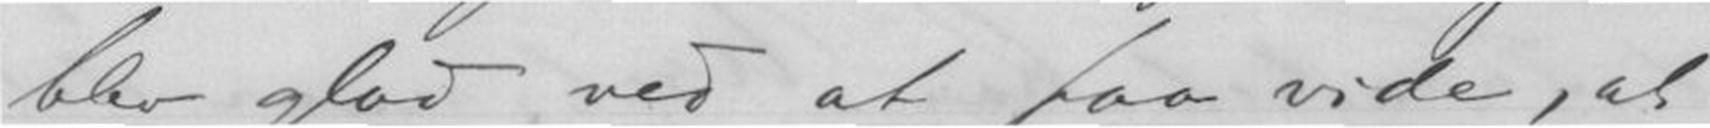blev glad ved at faa vide, at
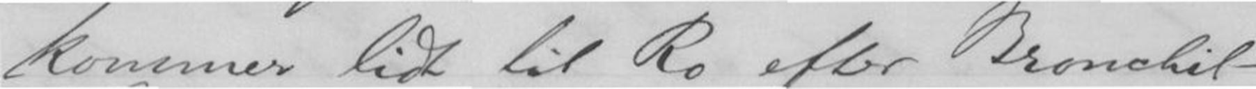kommer lidt til Ro efter Bronchit-
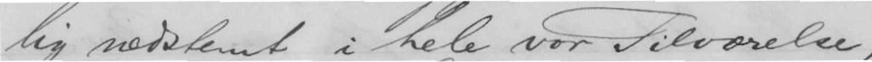lig nedstemt i hele vor Tilværelse,
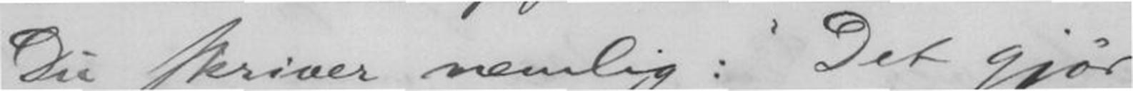Du skriver nemlig: "Det gjør
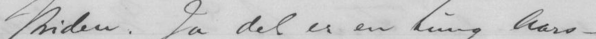striden. Ja det er en tung Aars-
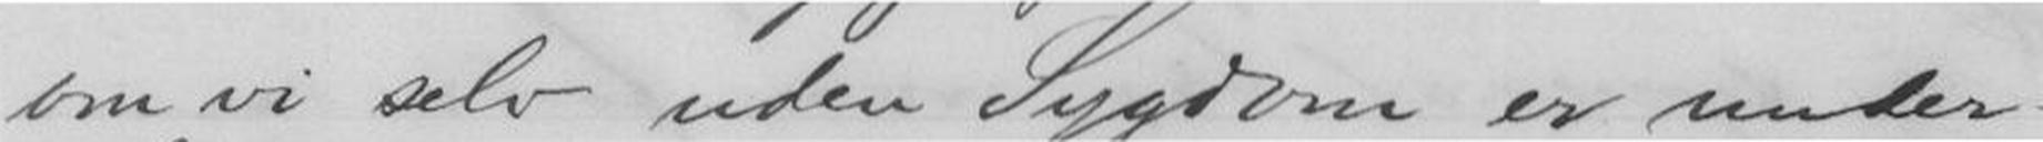om vi selv uden Sygdom er under-
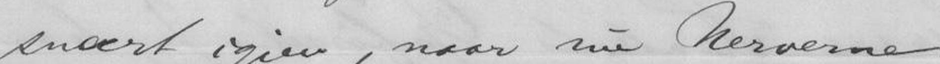snart igjen, naar nu Nærvene
